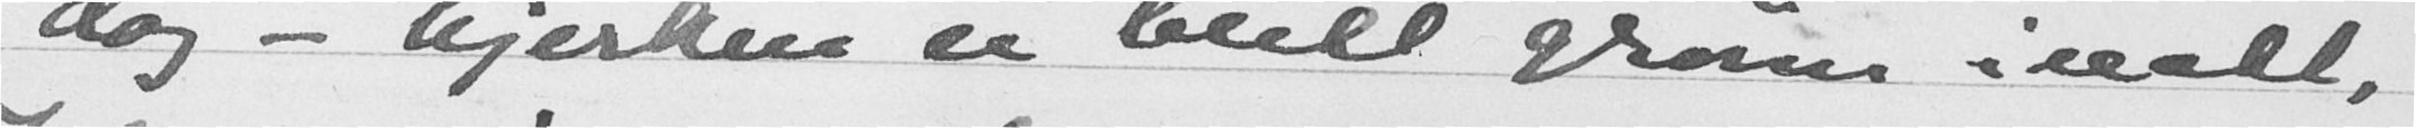dag - bjerken er blitt grønn inatt,
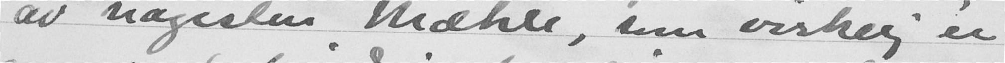av magister Mæhle, som virkelig er
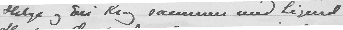Helge og Eli Krog sammen med Sigurd
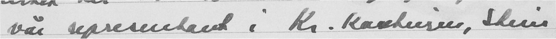vår representant i Kr. kastningen, Stein
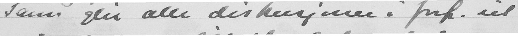Sånn glir alle diskusjone i forf. ut
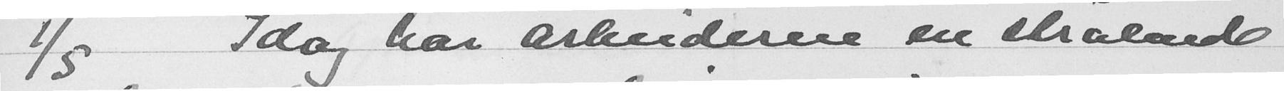1/5 Idag har arbeideren en strålende
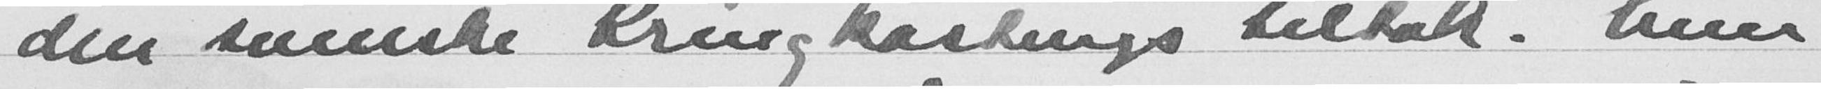den svenske Kringkastings tiltak. Men
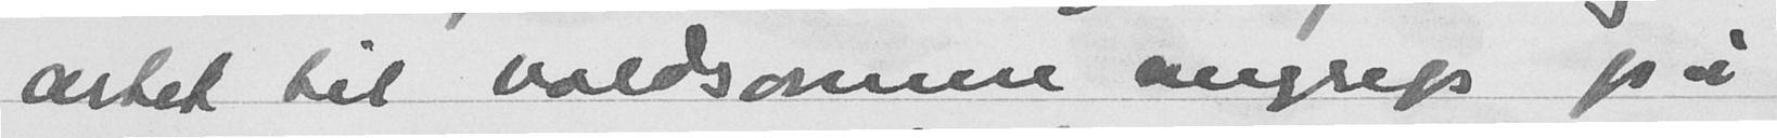artet til voldsomme angrep på
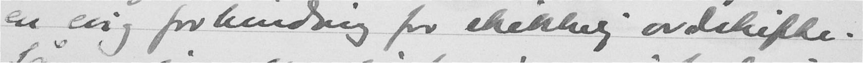en evig forhindring for skikkelig ordskifte.
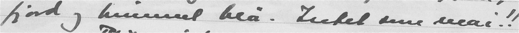fjord og himmel blå. Irtet som mai!
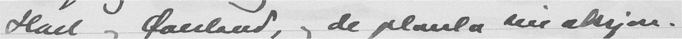Hoel og Øverland, og de planla sin aksjon.
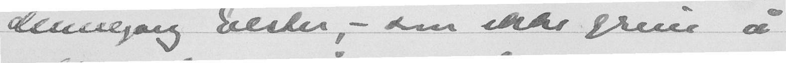dennegang Elster, - som ikke greier å
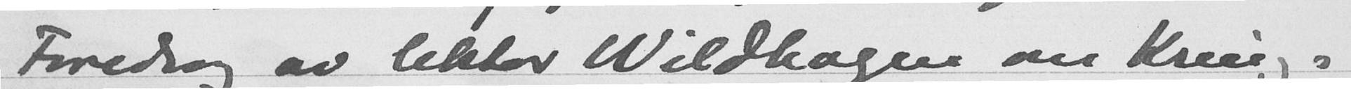Foredrag av lektor Wildhagen om Kring-
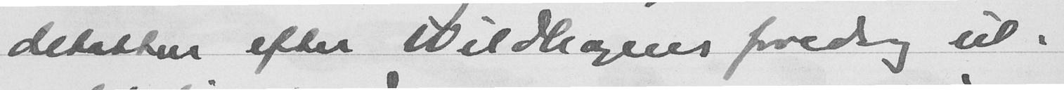debatten efter Wildhagens foredrag ut-
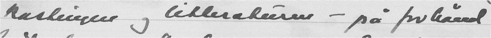kastingen og litteraturen - på forhånd
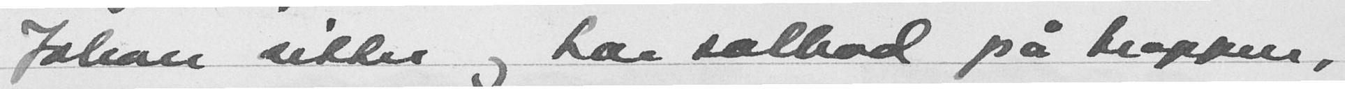Johan sitter og har solbad på trappen,
